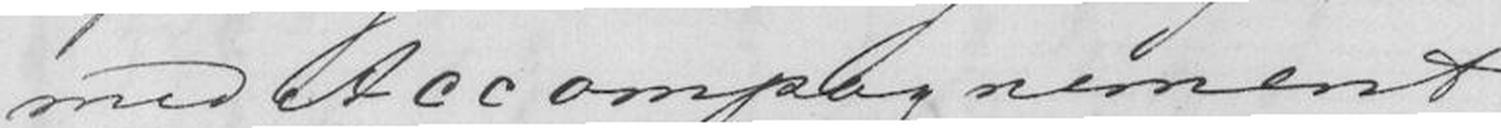med Accompagnement
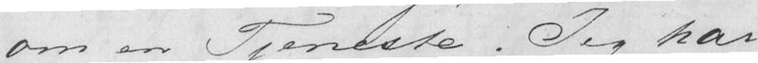om en Tjeneste. Jeg har
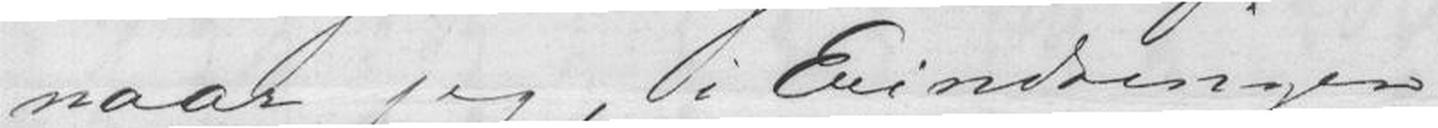naar jeg, i Erindringen
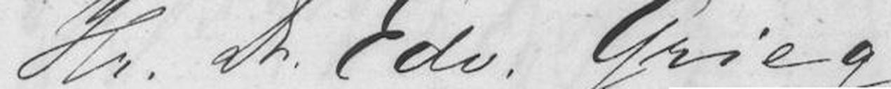Hr. Dr. Edv. Grieg
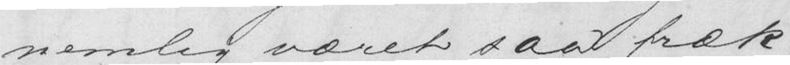nemlig været saa fræk
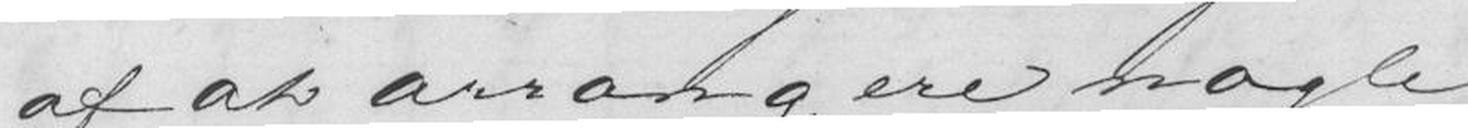af at arrangere nogle
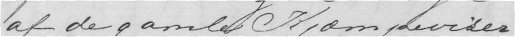af de gamle Kjæmpeviser
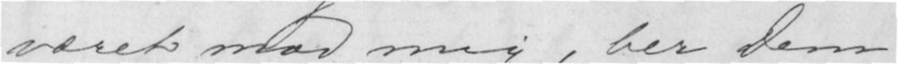været mod mig, ber Dem
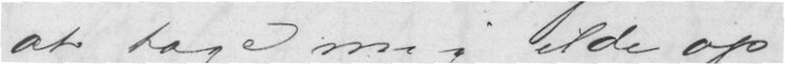at tage mig ilde op
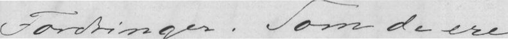Fordringer. Som de ere
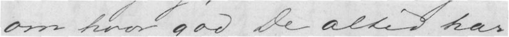om hvor god De altid har
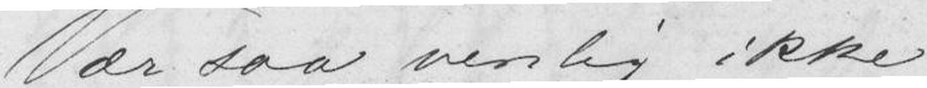Vær saa venlig ikke
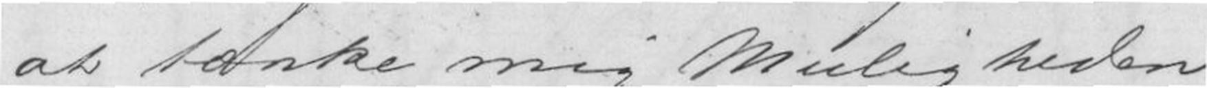at tænke mig Muligheden
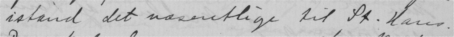istand det væsentlige til St. Hans.
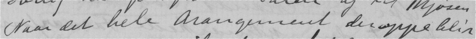Naar det hele Arangement deroppe blir
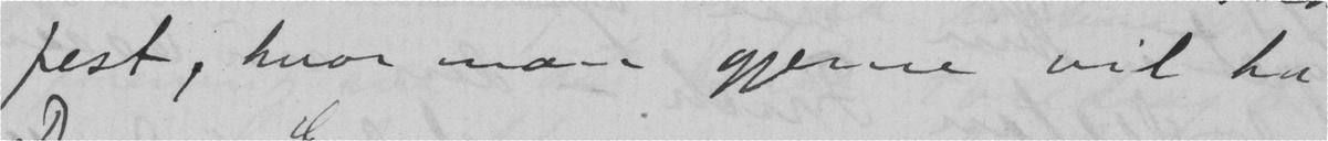fest, hvor man gjerne vil ha
